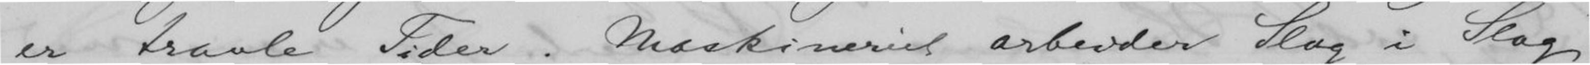er travle Tider. Maskineriet arbeider Slag i Slag
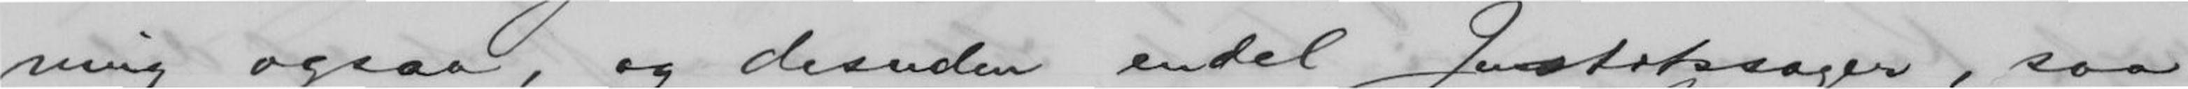ning ogsaa, og desuden endel Justitssager, saa
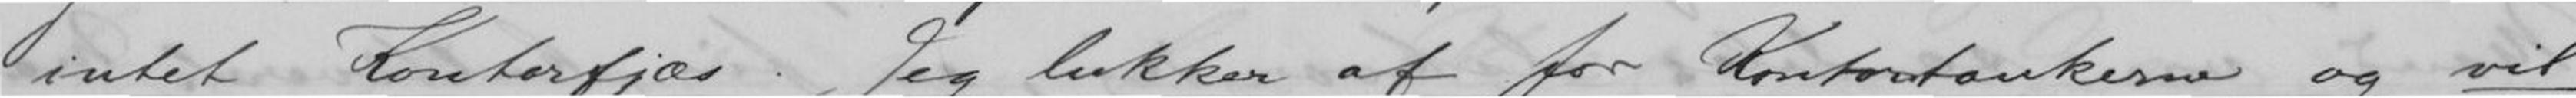intet Kontorfjæs. Jeg lukker af for Kontortankerne og vil
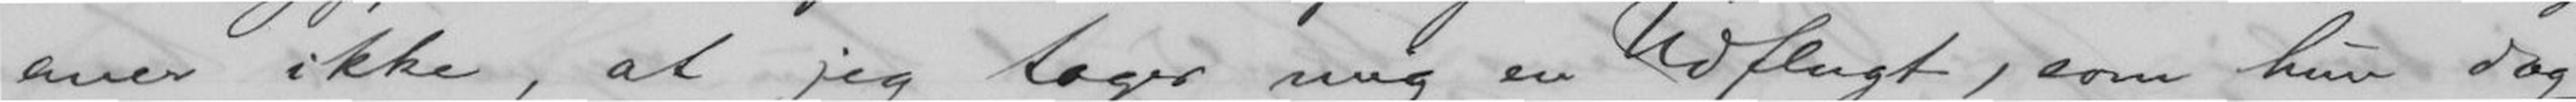aner ikke, at jeg tager mig en Udflugt, som hun dog
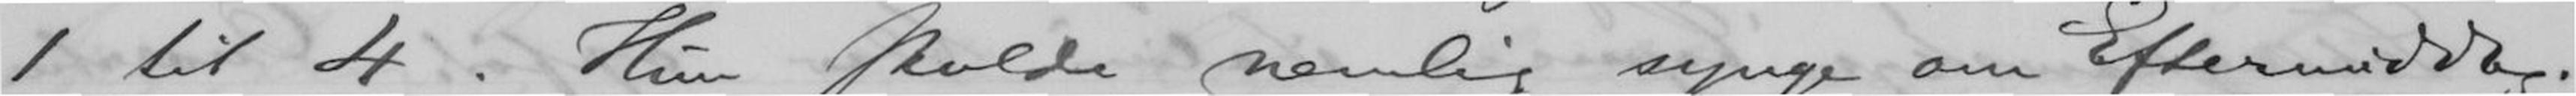1 til 4. Hun skulde nemlig synge om Eftermiddag.
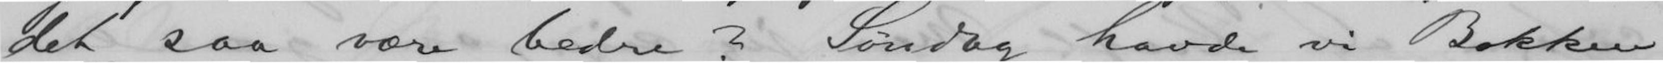det saa være bedre? Søndag havde vi Bokken
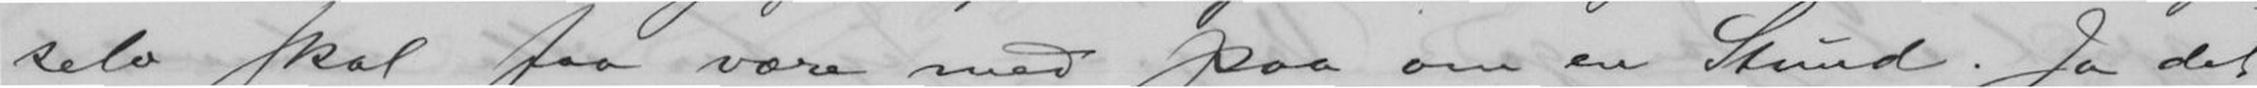selv skal faa være med paa om en Stund. Ja det
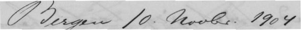Bergen 10 Novbr. 1904
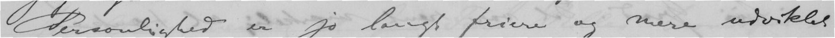Personlighed er jo langt friere og mere udviklet
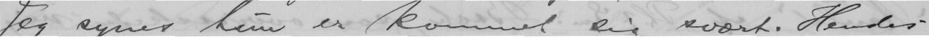Jeg synes hun er kommet sig svært. Hendes
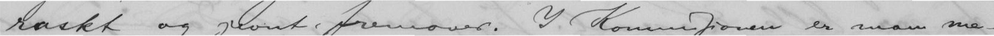raskt og jevnt fremover. I Kommissionen er man me-
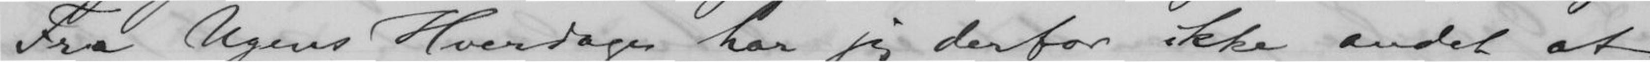Fra Ugens Hverdag har jeg derfor ikke andet at
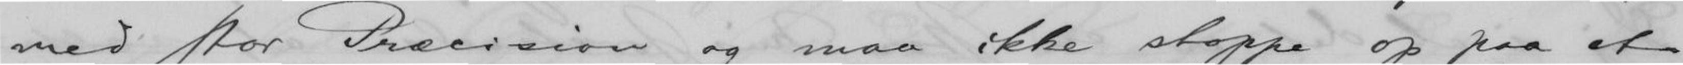med stor Præcision og maa ikke stoppe op paa et
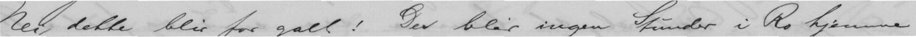Nei, dette blir for galt! Der blir ingen Stunder i Ro hjemme
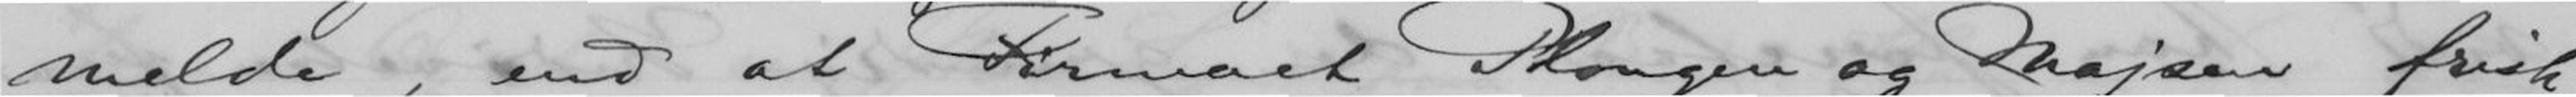melde, end at Firmaet Plougen og Majsen frisk
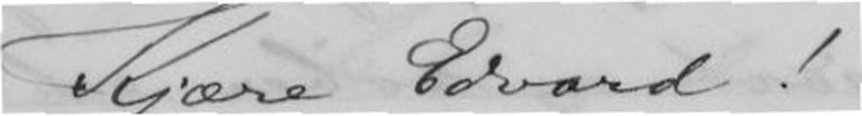Kjære Edvard!
